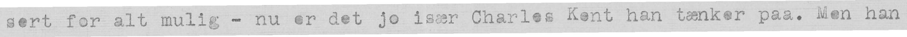sert for alt mulig - nu er det jo især Charles Kent han tænker paa. Men han
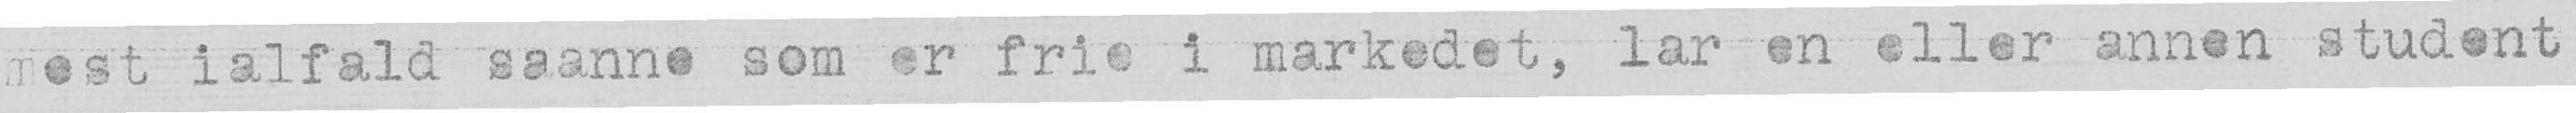mest ialfald saanne som er frie i markedet, lar en eller annen student
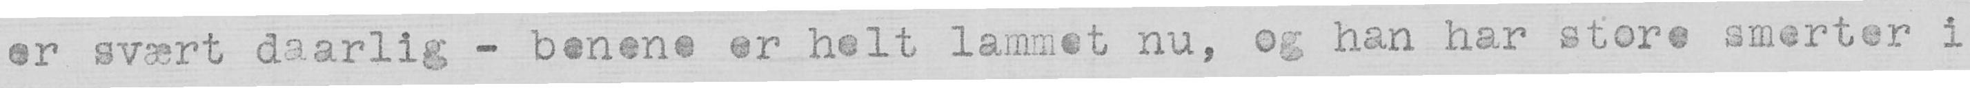er svært daarlig - benene er helt lammet nu, og han har store smerter i
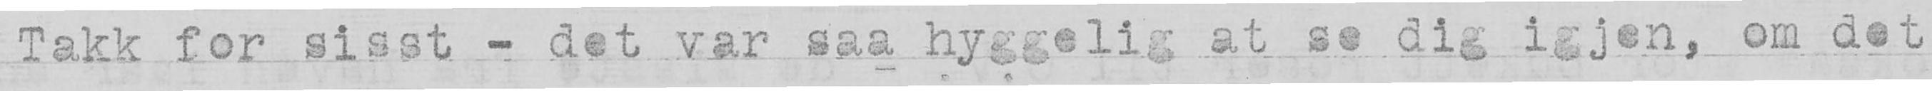Takk for sisst - det var saa hyggelig at se dig igjen, om det
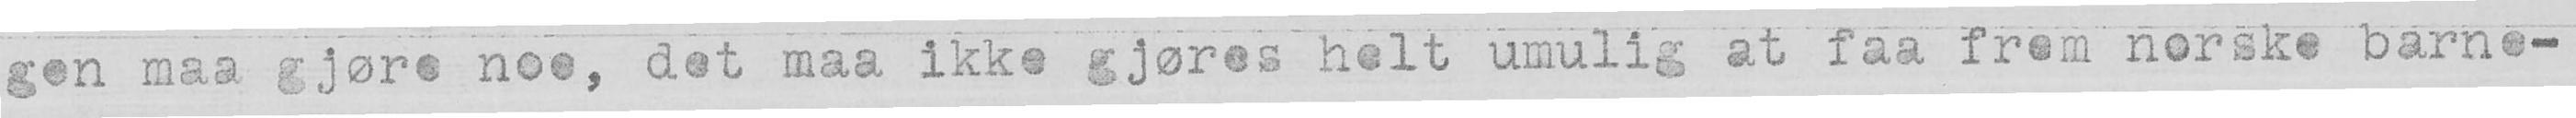gen maa gjøre noe, det maa ikke gjøres helt umulig at faa frem norske barne¬
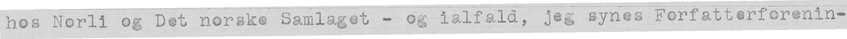hos Norli og Det norske Samlaget - og ialfald, jeg synes Forfatterferenin¬
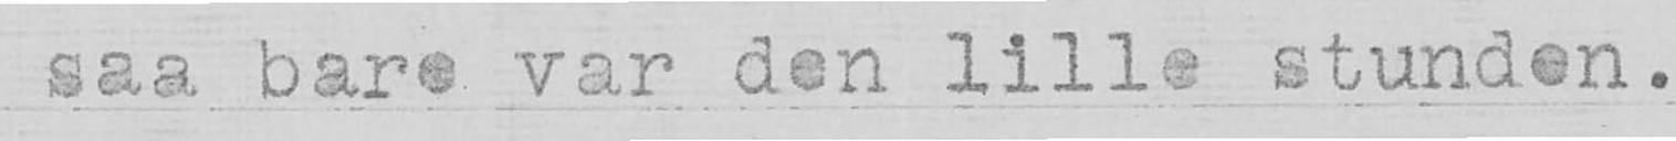saa bare var den lille stunden.
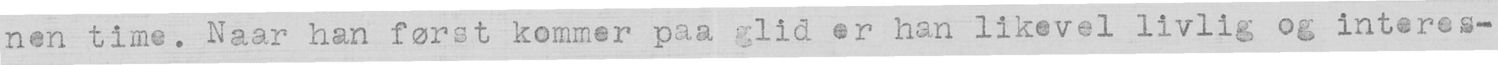nen time. Naar han først kommer paa glid er han likevel livlig og interes-
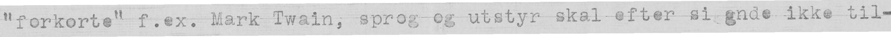"forkorte" f.ex. Mark Twain, sprog og utstyr skal efter si gnde ikke til-
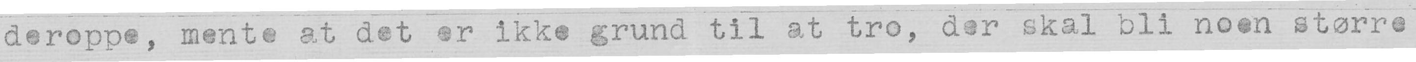deroppe, mente at det er ikke grund til at tro, der skal bli noen større
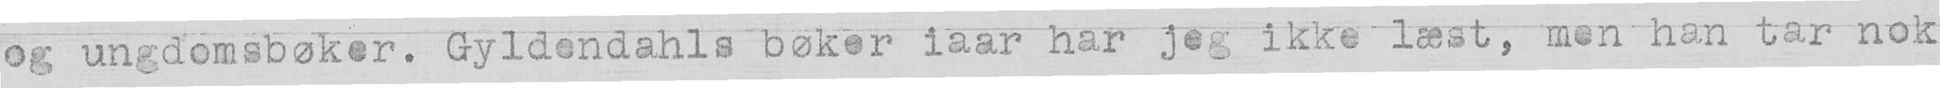og ungdomsbøker. Gyldendahls bøker iaar har jeg ikke læst, men han tar nok
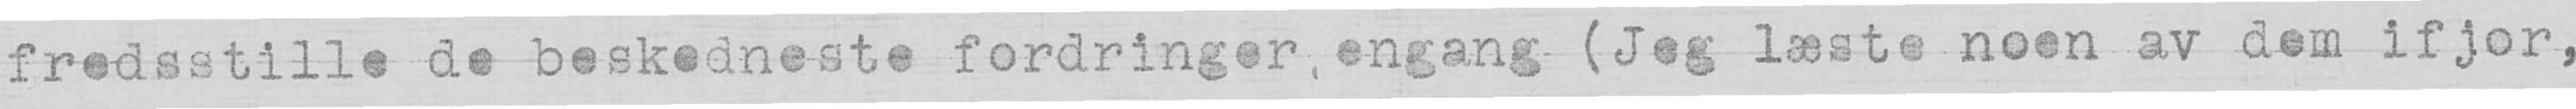fredsstille de beskedneste fordringer engang (Jeg læste noen av dem ifjor,
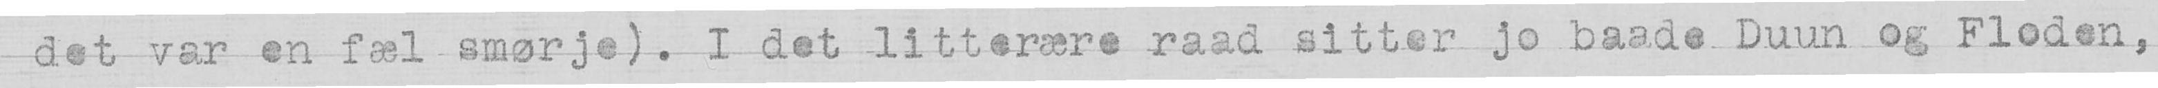det var en fæl smørje). I det litterære raad sitter jo baade Duun og Floden,
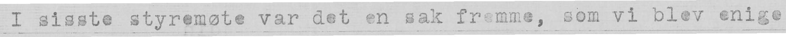I sisste styremøte var det en sak fremme, som vi blev enige
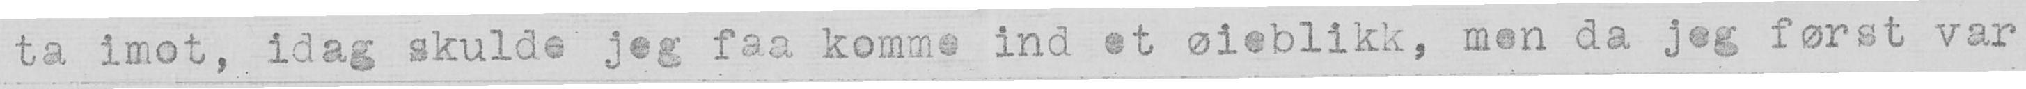ta imot, idag skulde jeg faa komme ind et øieblikk, men da jeg først var
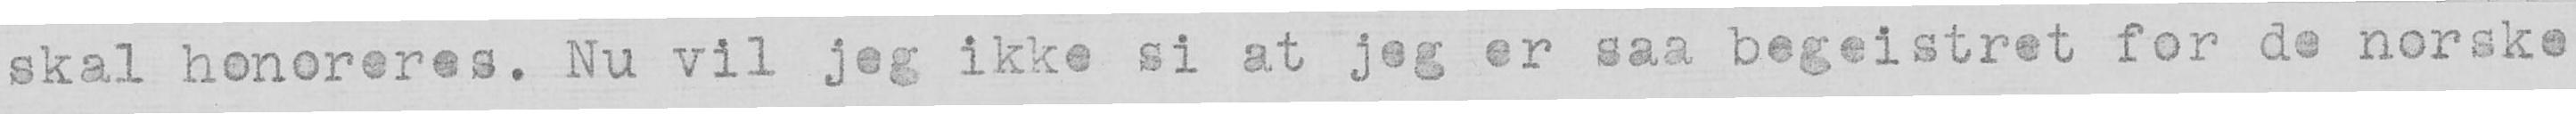skal honoreres. Nu vil jeg ikke si at jeg er saa begeistret for de norske
